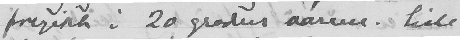foregikk i 20 graders varme. Siste
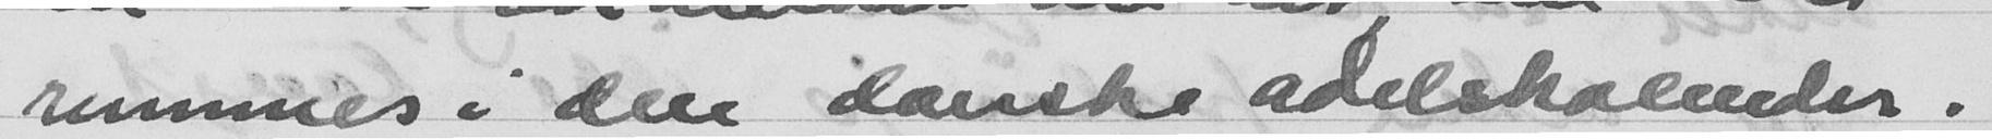rummer i den danske adelskalender.
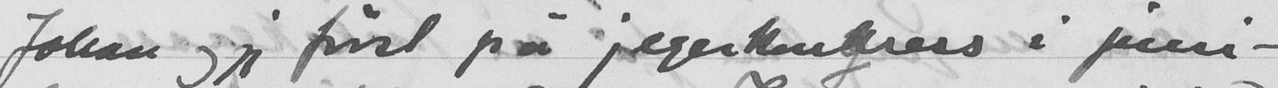Johan og jeg først på Jegerkongress i jeen-
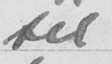til
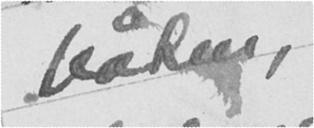båten,
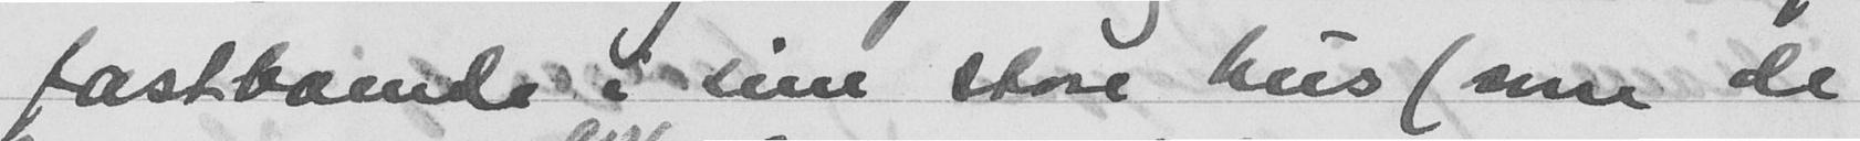fastboende i sine store hus (som de
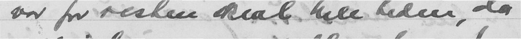Jeg var for resten skral hele tiden, da
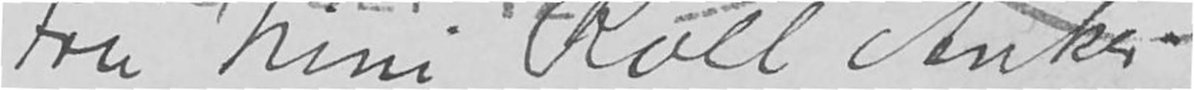Fru Nini Roll Anker
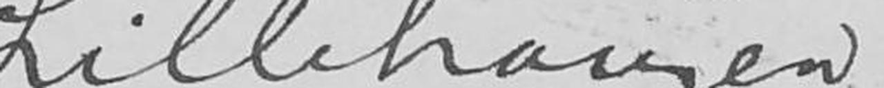Lillehaugen
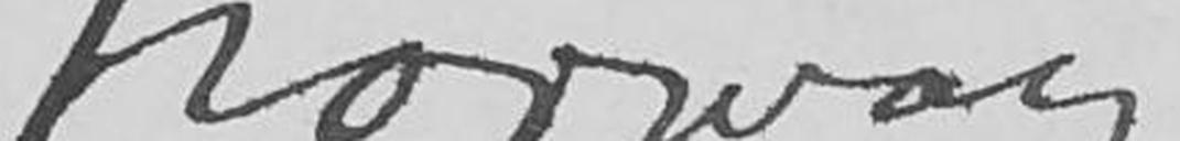Norway
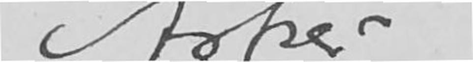Asker
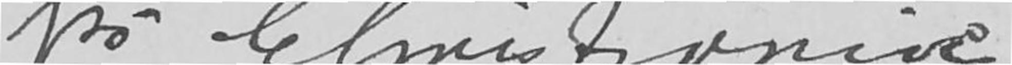På Elmistronive
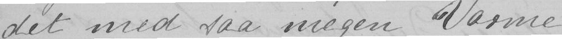det med saa megen Varme,
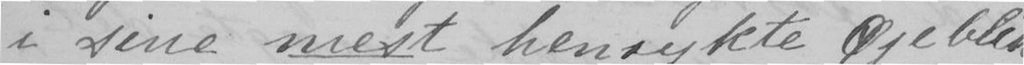i sine mest henrykte Øjeblik-
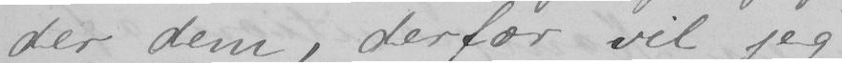der dem, derfor vil jeg
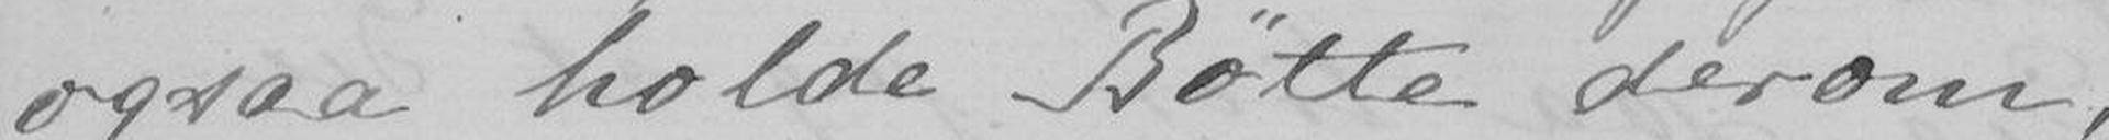ogsaa holde Bøtte derom,
